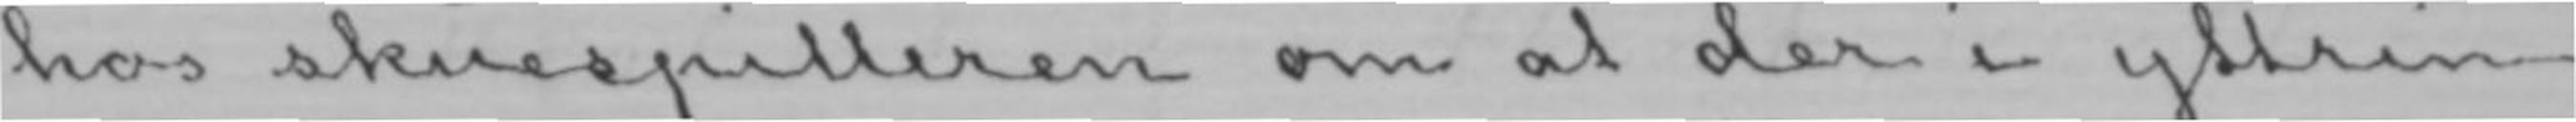hos skuespilleren om at der i yttrin-
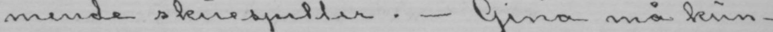mende skuespiller.- Gina må kun-
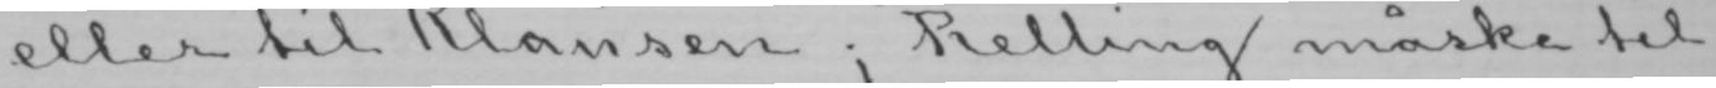eller til Klausen; Relling måske til
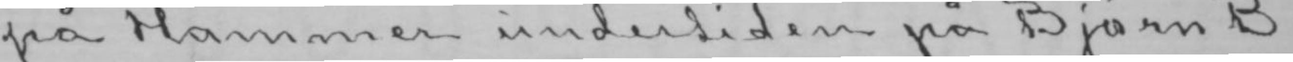på Hammer undertiden på Bjørn B.
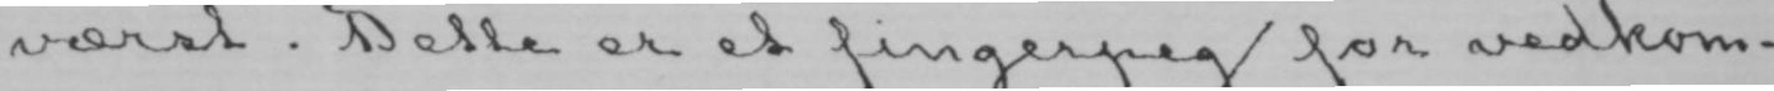værst. Dette er et fingerpeg for vedkom-
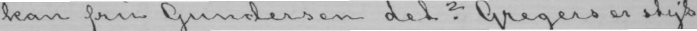kan fru Gundersen det? Gregers er styk-
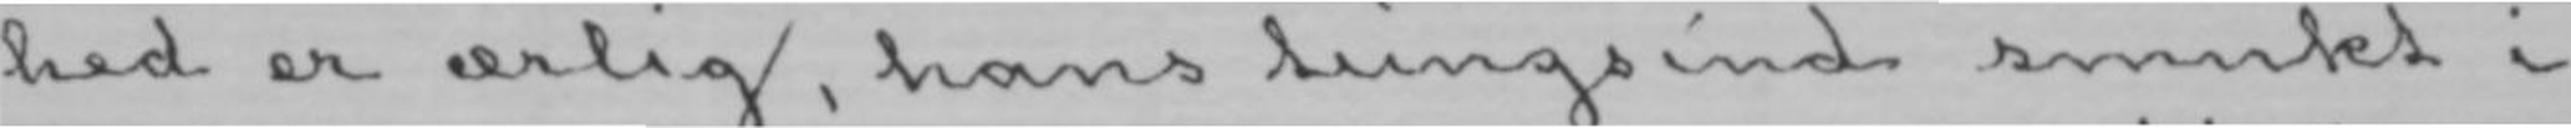hed er ærlig, hans tungsind smukt i
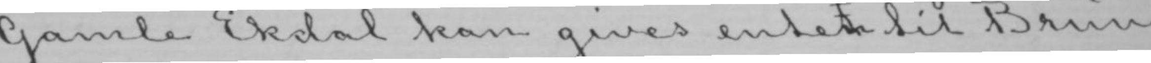Gamle Ekdal kan gives entetn til Brun
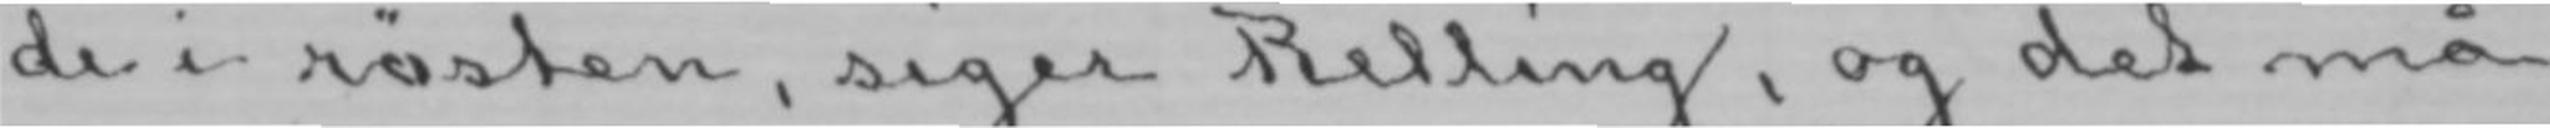de i røsten, siger Relling, og det må
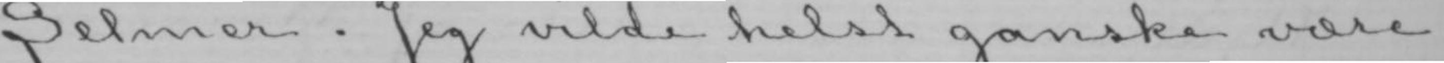Selmer. Jeg vilde helst ganske være
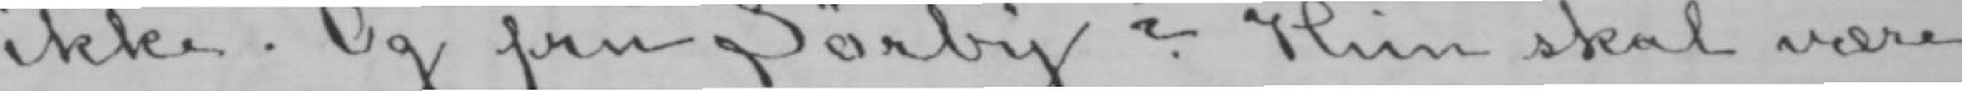ikke. Og fru Sørby? Hun skal være
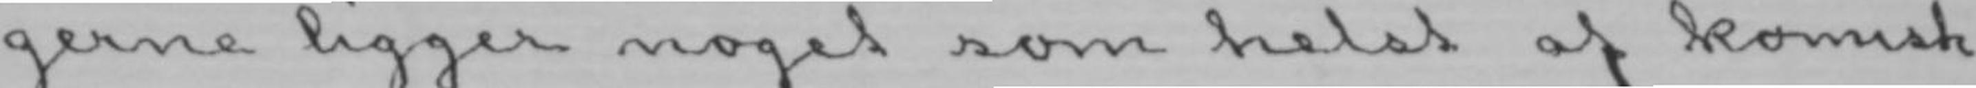gerne ligger noget som helst af komisk
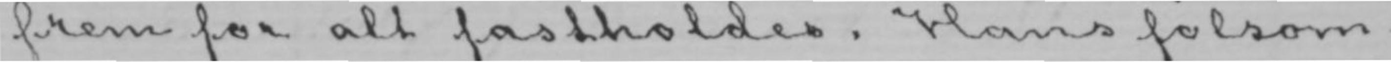frem for alt fastholdes. Hans følsom-
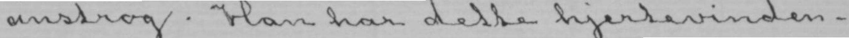anstrøg. Han har dette hjertevinden-
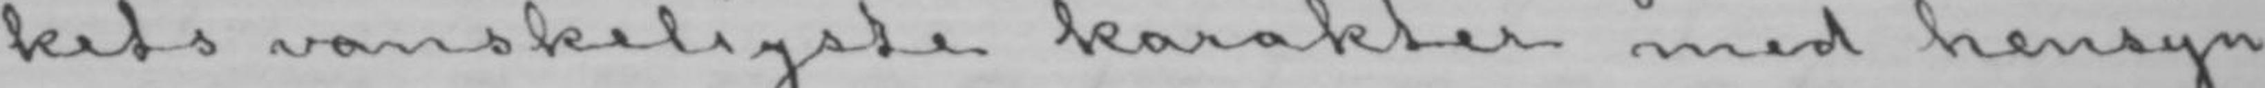kets vanskeligste karakter med hensyn
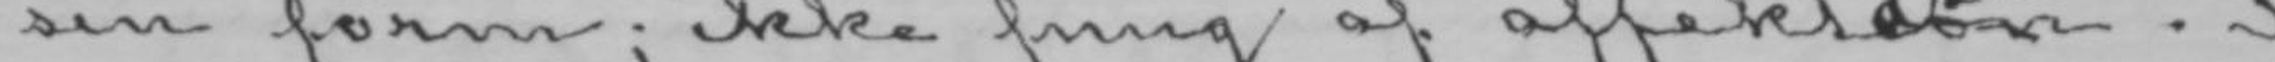sin form; ikke fnug af affektation. I
